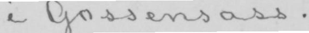i Gossensass.
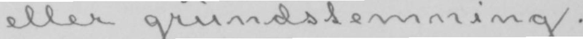eller grundstemning.
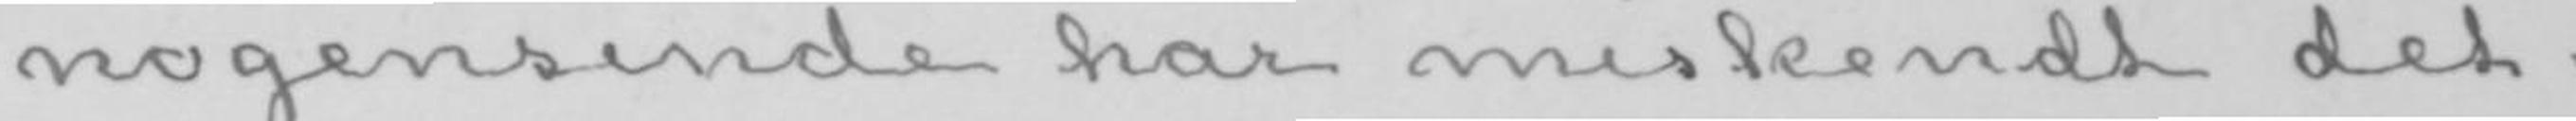nogensinde har miskendt det-
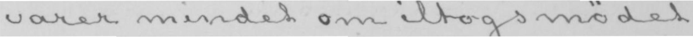varer mindet om iltogsmødet
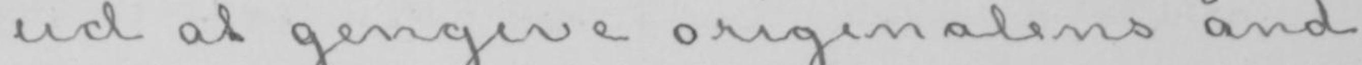ud at gengive originalens ånd
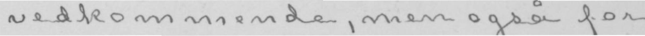vedkommende, men også for
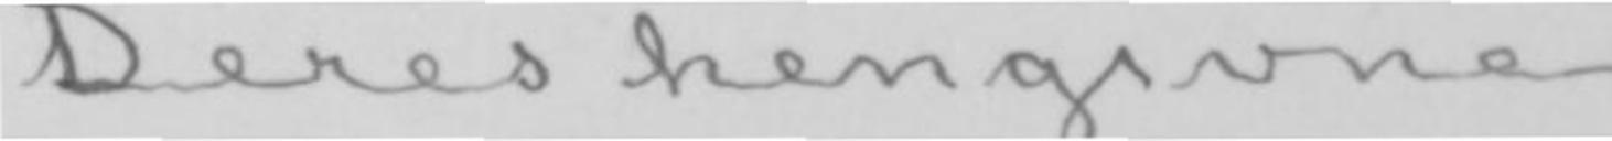Deres hengivne
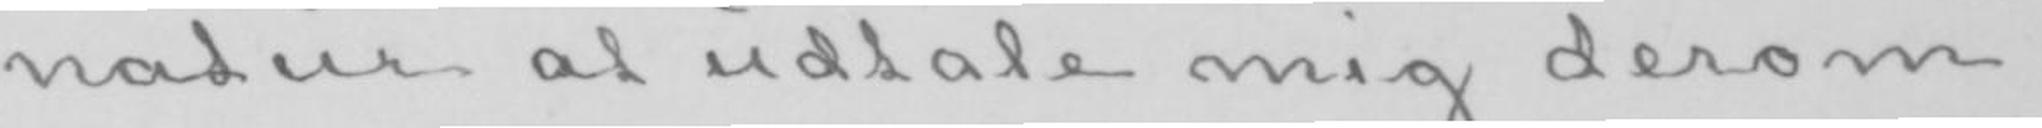natur at udtale mig derom.
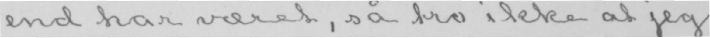end har været, så tro ikke at jeg
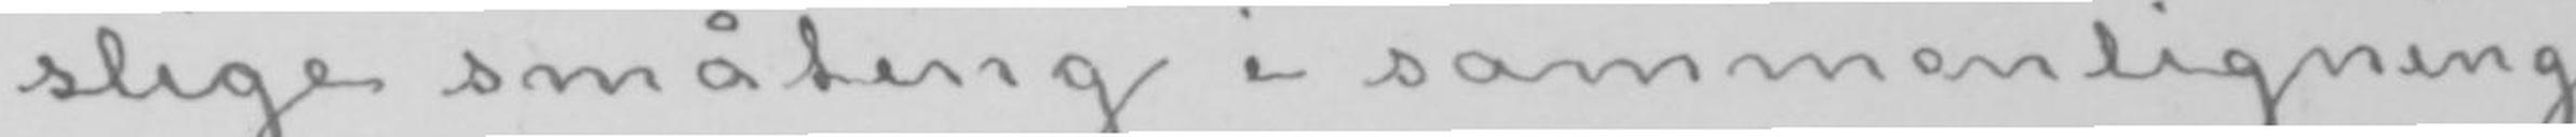slige småting i sammenligning
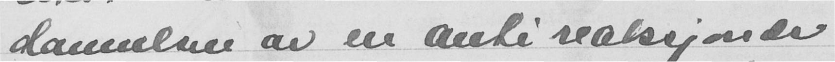dannelsen av en anti reaksjonær
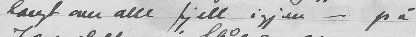langt over alle fjell igjen - på
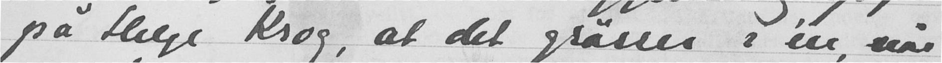på Helge Krog, at det grøsser i en, når
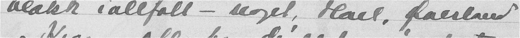blokk iallfall - noget, Hoel, Øverland
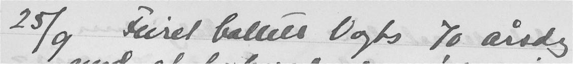25/9 Feiret Collett Vogts 70 årsdag
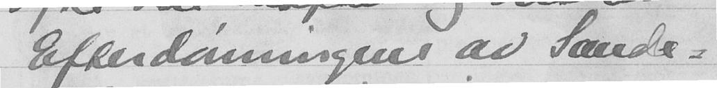Efterdønningen av Sande-
